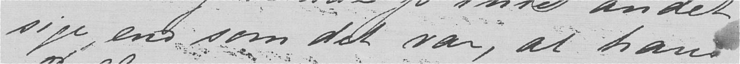sige end som det var, at hans
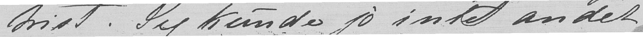trist. Jeg kunde jo intet andet
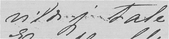vilde jeg tale
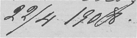22/4 1908.
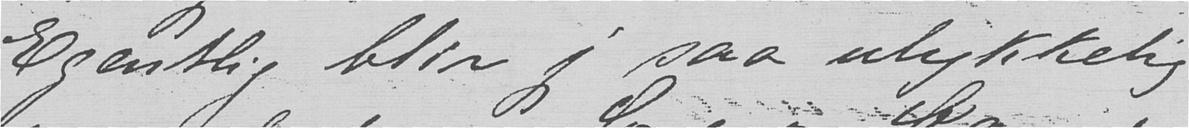Egentlig blir jeg saa ulykkelig
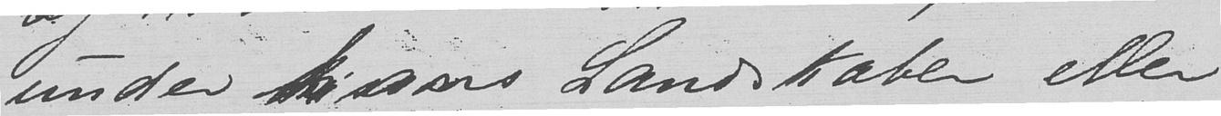under hans Landskaber eller
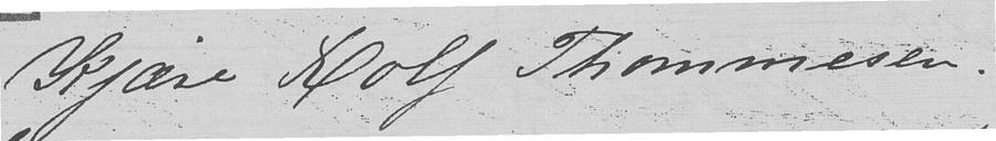Kjære Rolf Thommesen.
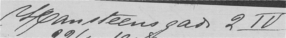Hansteensgade 2 IV
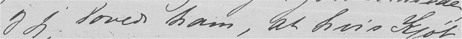og jeg lovede ham, at hvis Kjøb
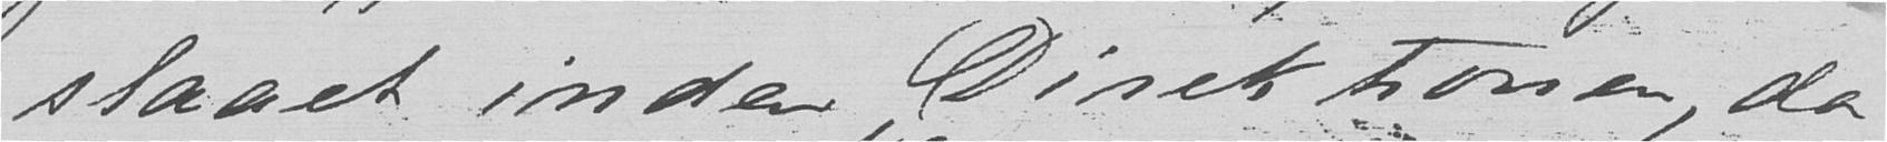slaaet inden Direktionen, da
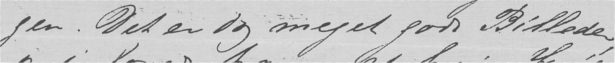gen. Det er dog meget gode Billeder
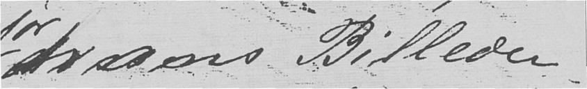hans Billeder.
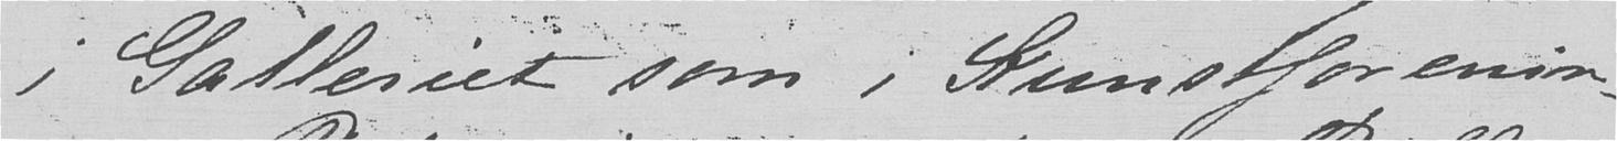i Galleriet som i Kunstforenin-
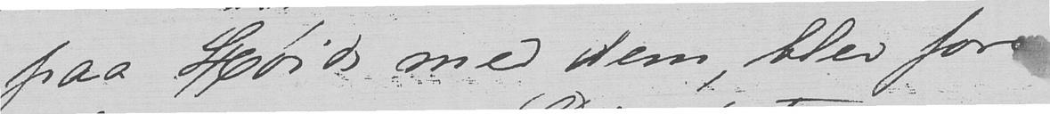paa Høide med dem, blev fore-
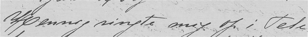Hennig ringte mig op i Tele-
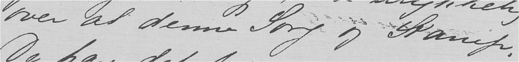over al denne Sorg og Kamp.
